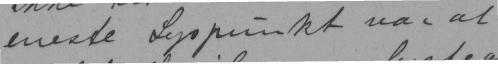eneste Lyspunkt var at
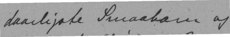daarligste Smaabarn og
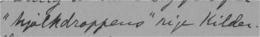"Mjølkdroppens" rige Kilder.
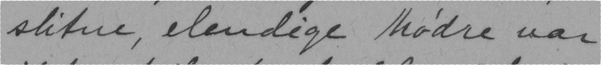slitne, elendige mødre var
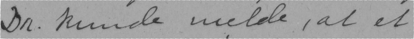Dr. kunde melde, at et
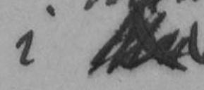i
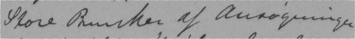Store Bunker af Ansøgninger
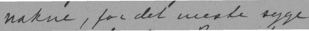nakne, for det meste syge
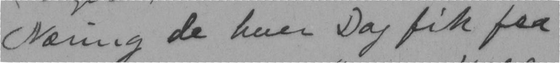Næring de hver Dag fik faa
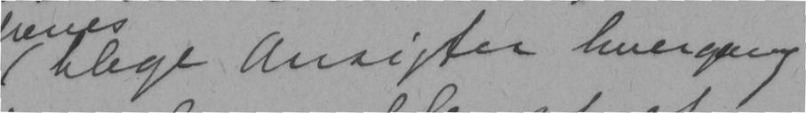ulege Ansigter hvergang
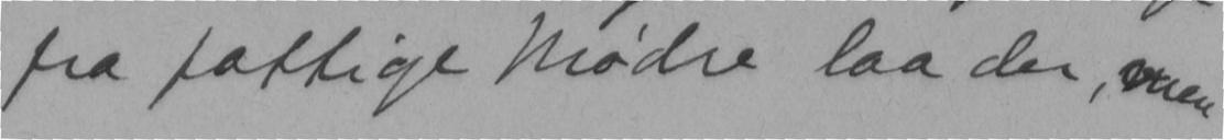fra fattige mødre laa der, men
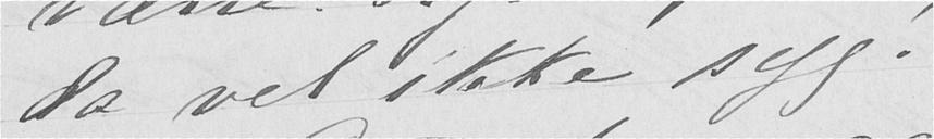da vel ikke syg?
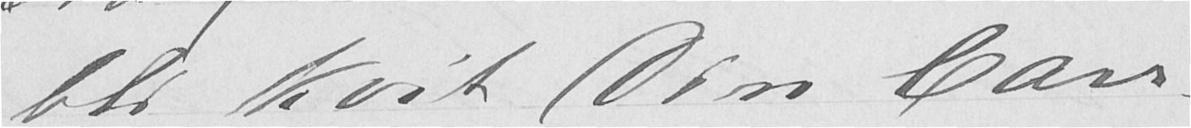bli kvit Din Car-
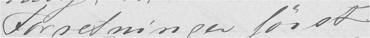Forretninger først
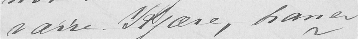være. Kjære, han er
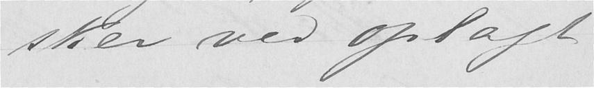sker ved oplagt
